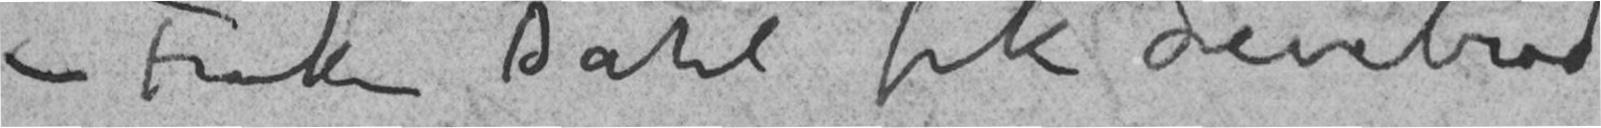– Frøken Dahl fik Levebrød
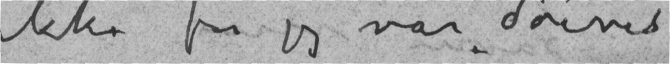ikke for jeg var døenes
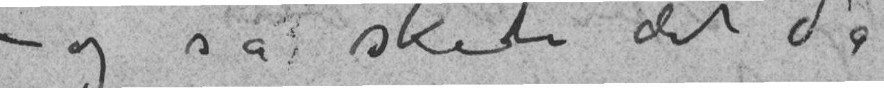– og så skete det da
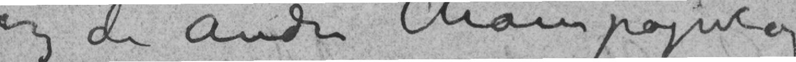og de andre Champagne
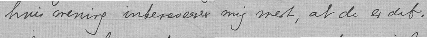hvis mening interssserer mig mest, at de er det.
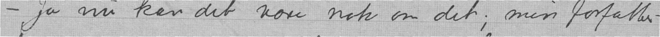- Ja nu kan det være nok om det; min forfatter-
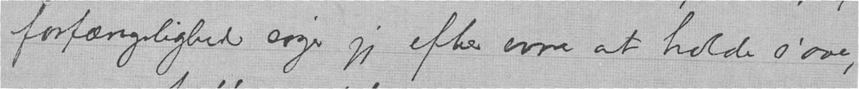forfængelighet søger jeg efter evne at holde i ave,
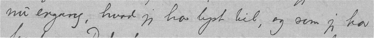nu engang, hvad jeg har lyst til, og som jeg har
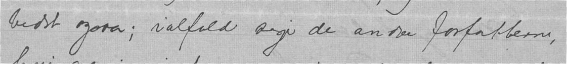bedst ogsaa; ialfald siger de andre forfatterne,
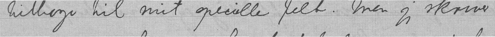tilbage til mit specielle felt. Men jeg skriver
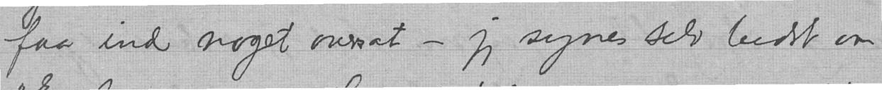faa ind noget oversat - jeg synes selv bedst om
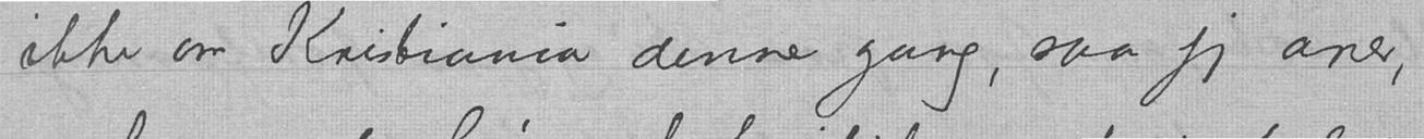ikke om Kristiania denne gang, saa jeg aner,
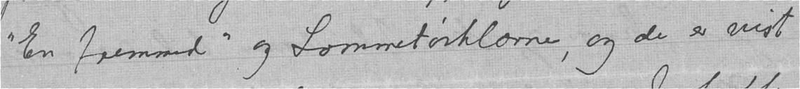"En fremmed" og Lommetørklærne, og de er vist
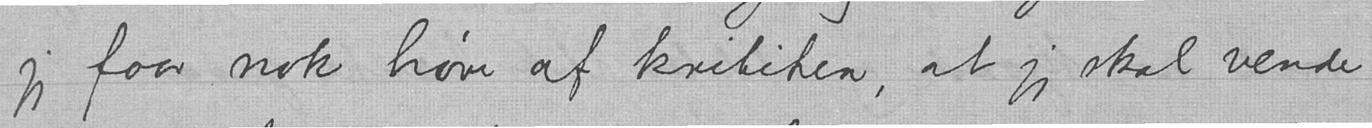jeg faar nok høre af kritiken, at jeg skal vende
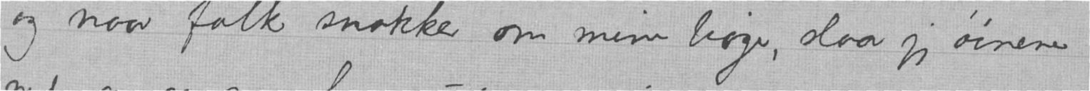og naar folk snakker om mine bøger, slaar jeg øinene
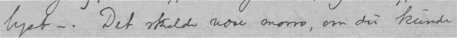lyst -. Det skulde være morro, om du kunde
